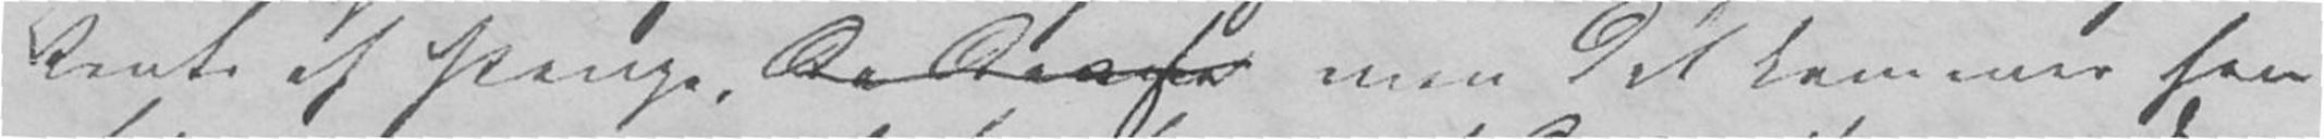Rente af Penge, men det kommer han
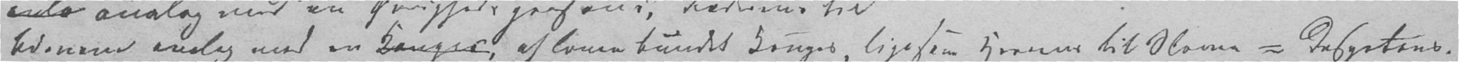Sønnens endog med en Konges, af Love bundet Konges, ligesom Herrens til Slaven = Despotens.
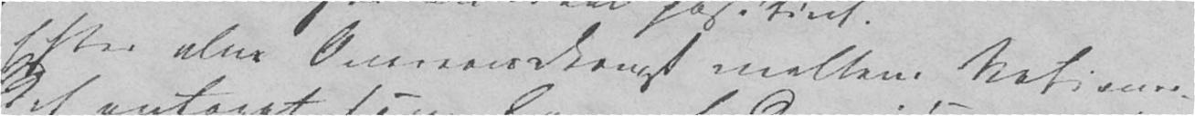Efter alm Overeenskomst mellem Nationer-
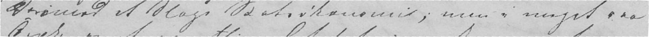Derimod et Slags Statsøkonomie; men i meget raa
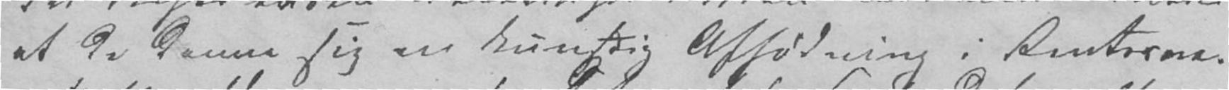at de danne sig en kunstig Affødning i Renterne.
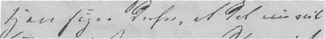Han siger derfor, at det vis vil
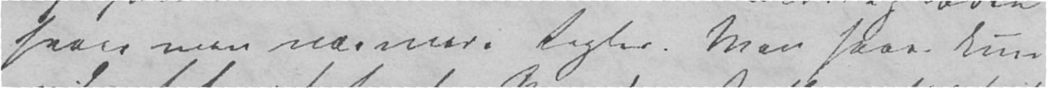faaer man nærmere Regler. Man faar kun
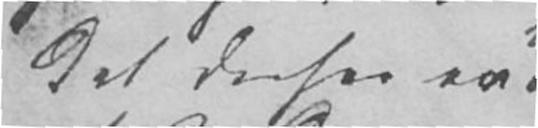det derfor er
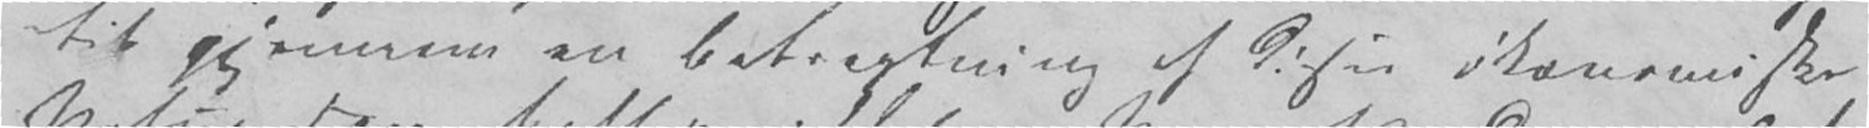til gjennem en Betragtning af disses økonomiske
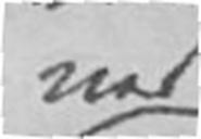ved
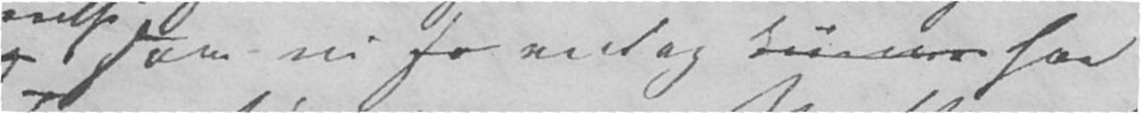som vi endog faa
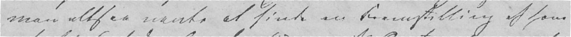man altsaa vente at finde en Fremstilling af hans
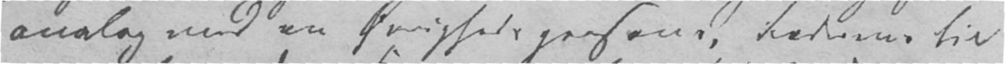ele analog med en Øvrighedsperson, Faderens til
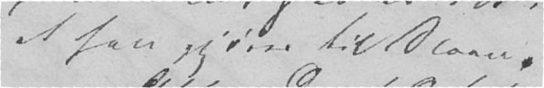at han gjøres til Slave.
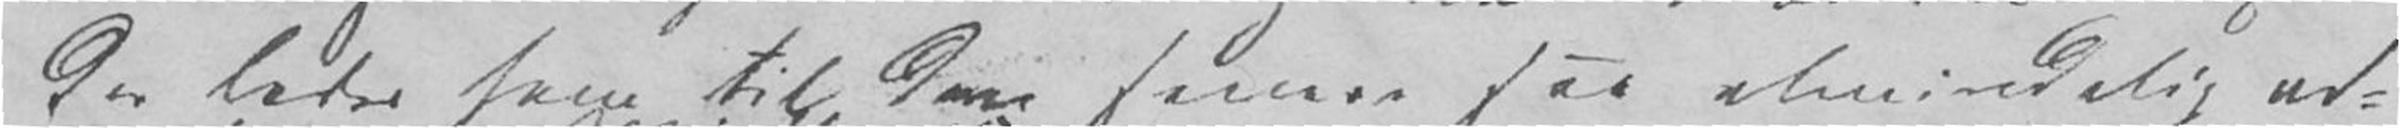der leder ham til den samme saa almindelig ad-
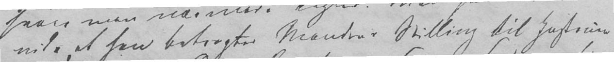vide, at han betragter Mandens Stilling til Hustruen
